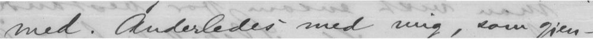med. Anderledes med mig, som gjen-
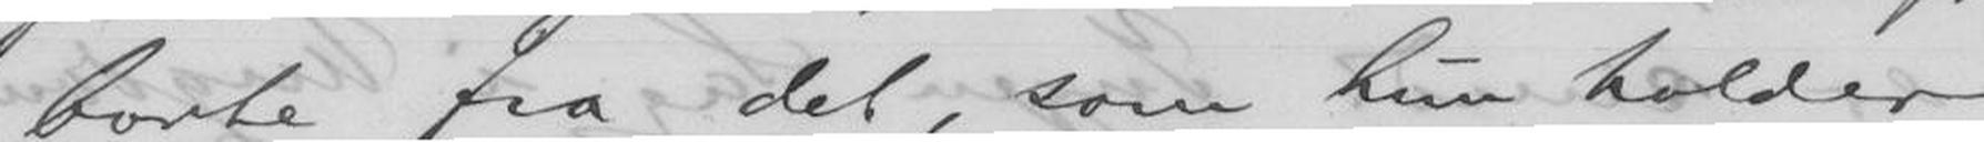borte fra det, som hun holder
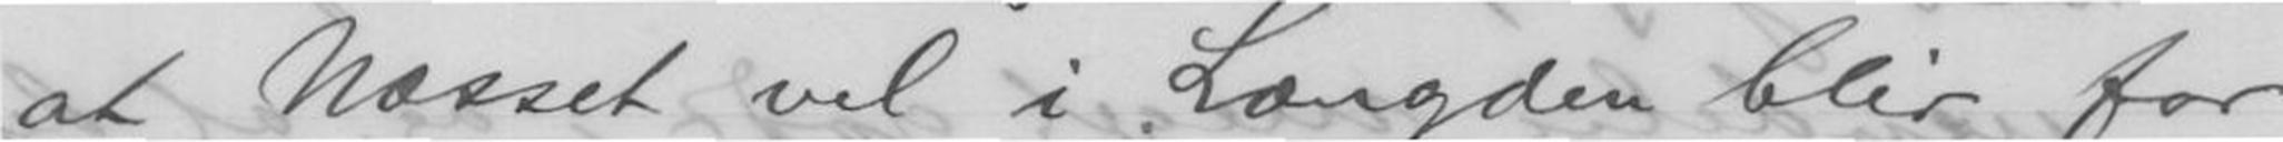at Næsset vel i Længden blir for
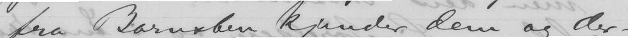fra Barnsben kjender dem og der-
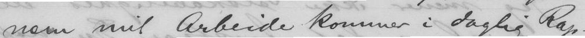nem mit Arbeide kommer i daglig Rap-
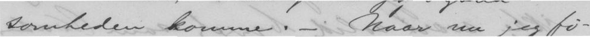somheden komme. - Naar nu jeg fø-
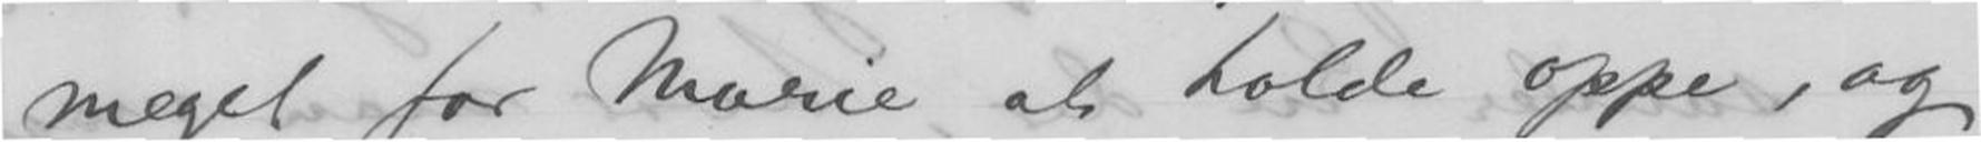meget for Marie at holde oppe, og
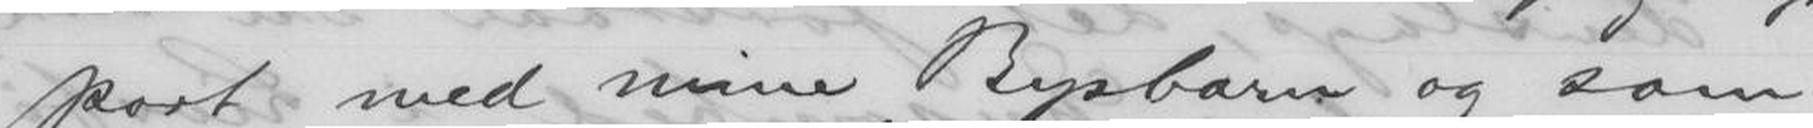port med mine Bysbarn og som
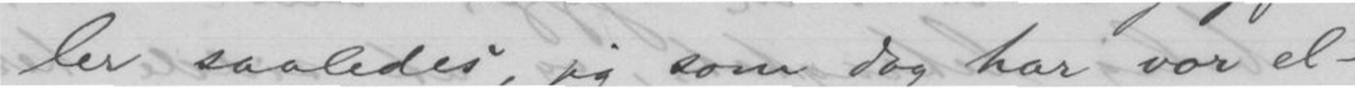ler saaledes, jeg som dog har vor el-
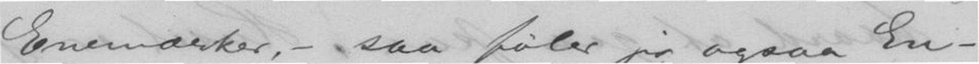Enemærker, - saa føler jeg ogsaa En-
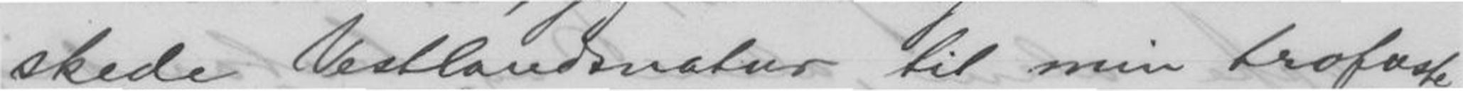skede Vestlandsnatur til min trofaste
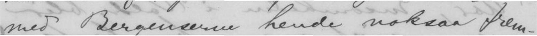med Bergenserne hende noksaa frem-
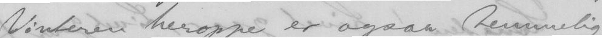Vinteren heroppe er ogsaa temmelig
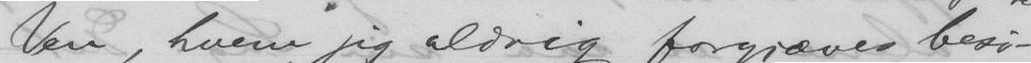Ven, hvem jeg aldrig forgjæves besø-
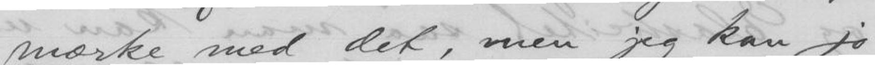mærke med det, men jeg kan jo
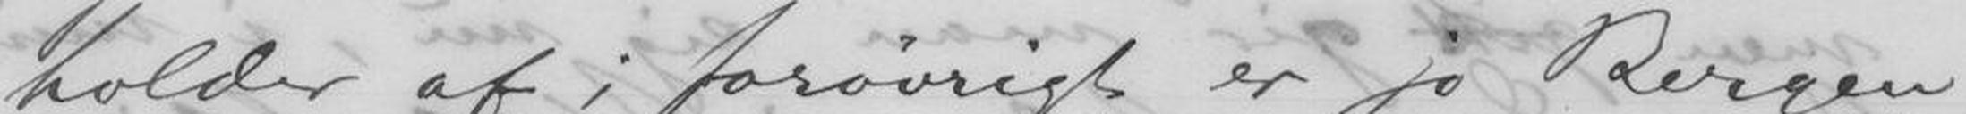holder af; forøvrigt er jo Bergen
